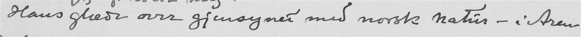Hans glæde over gjensynet med norsk natur - i Aren
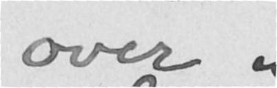over
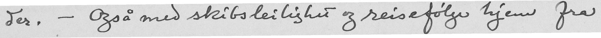der. - Også med skibsleilighet og reisefølge hjem fra
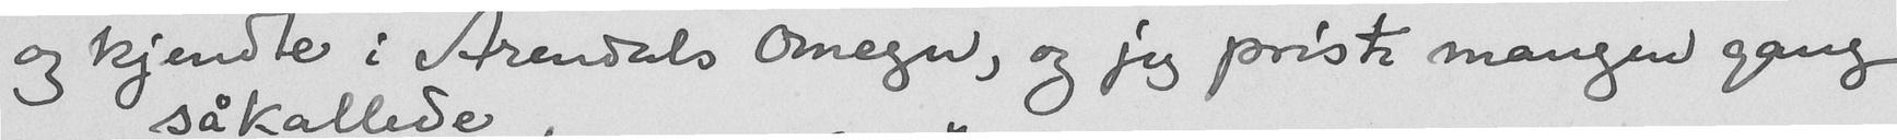og kjendte i Arendals omegn, og jeg priste mangen gang
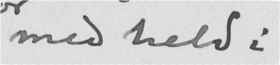med held i
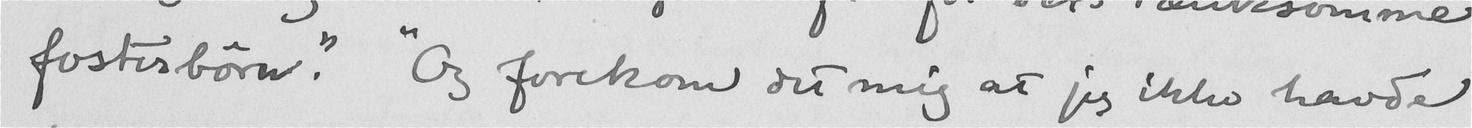fosterbørn." "Og forekom det mig at jeg ikke havde
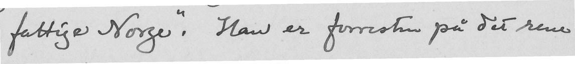fattige Norge." Han er forresten på det rene
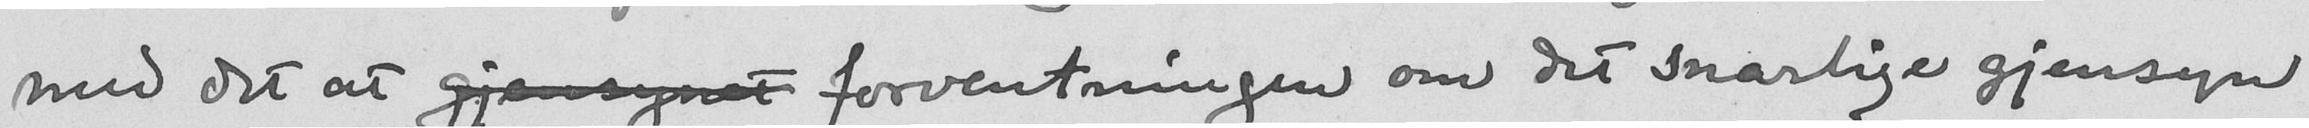med det at gjensynet forventningen om det snarlige gjensyn
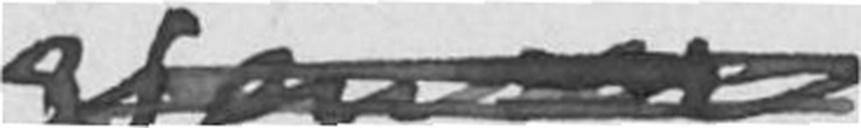gjænsyn
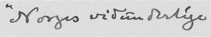"Norges vidunderlige
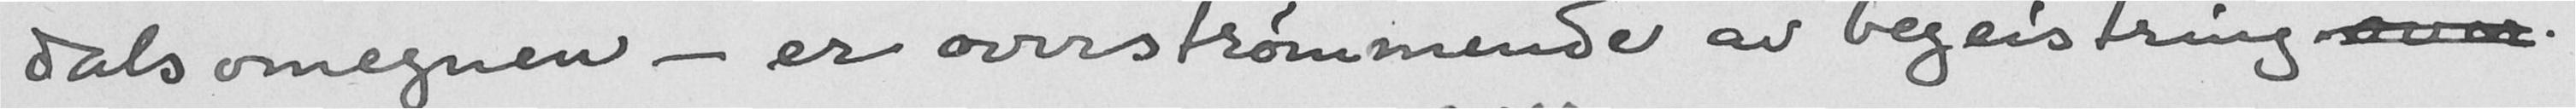dals omegnen - er overstrømmende av begeistring over
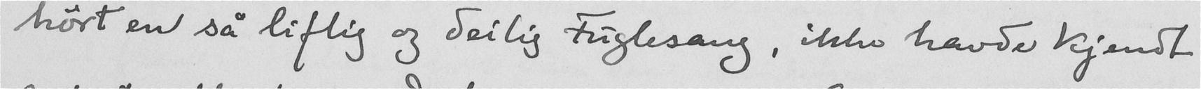hørt en så liflig og deilig Fuglesang, ikke havde kjendt
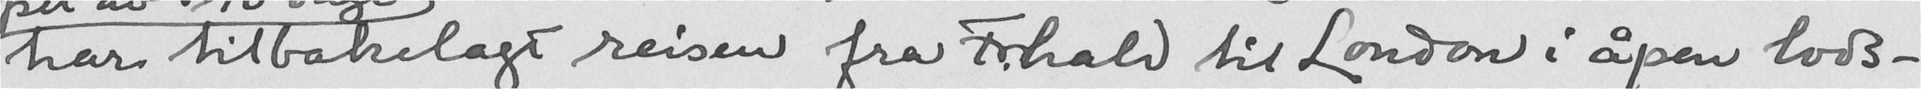har tilbakelagt reisen fra Fr.hald til London i åpen lods-
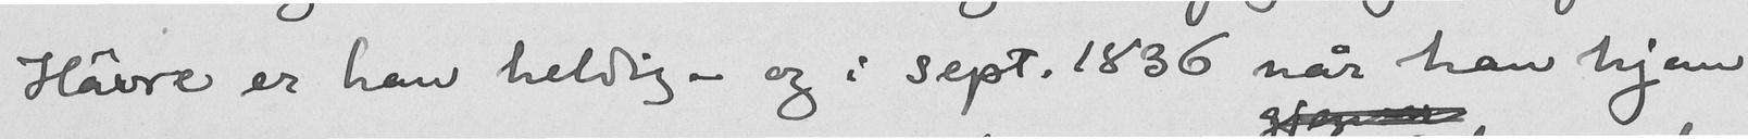Hâvre er han heldig - og i sept. 1836 når han hjem
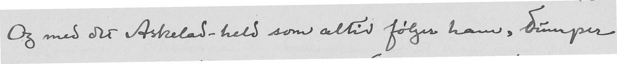Og med det Askelad-held som altid følger ham, dumper
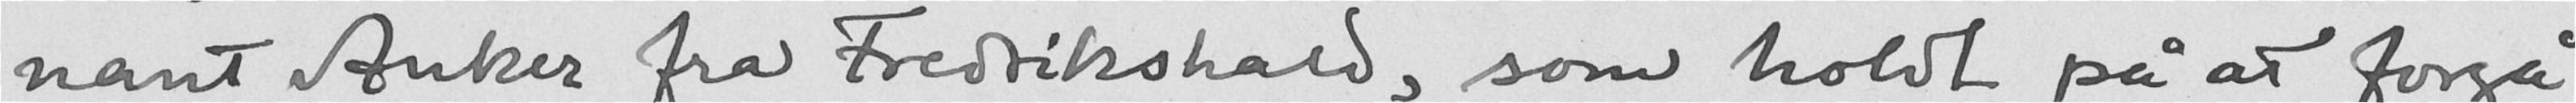nant Anker fra Fredrikshald, som holdt på at forgå
